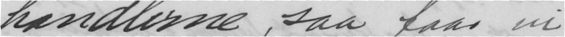handlerne, saa faar vi
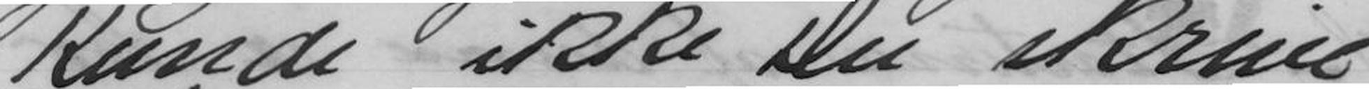Kunde ikke Du skrive
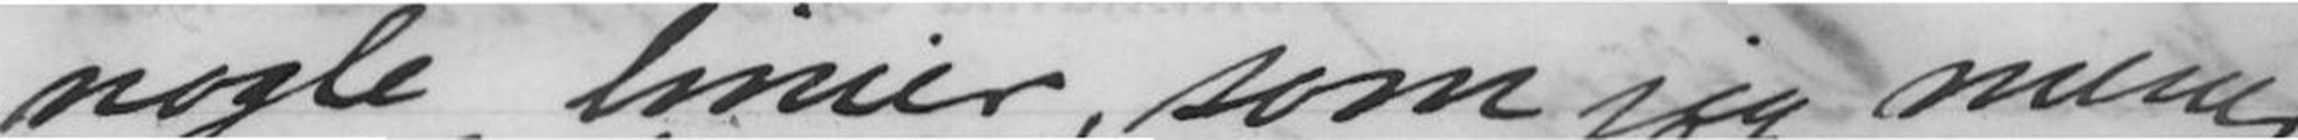nogle linier, som jeg mener
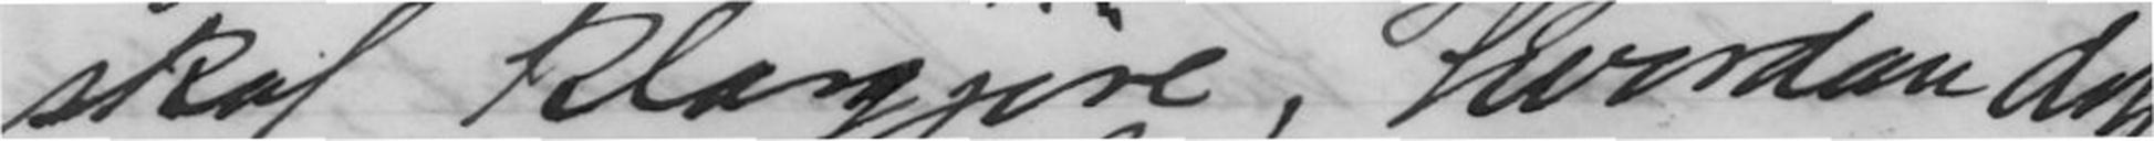skal klargjøre, hvordan denne
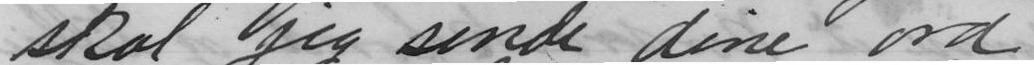skal jeg sende dine ord
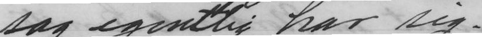sag egentlig har sig.
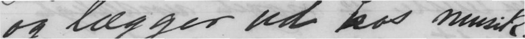og lægger ud hos musik-
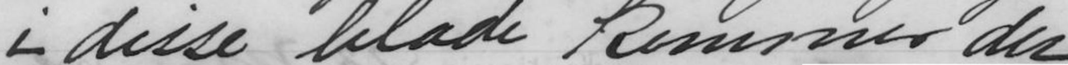i disse blade kommer der
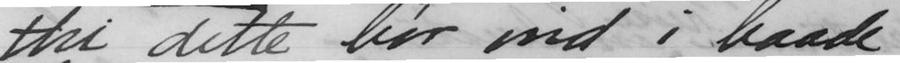thi dette bør ind i baade
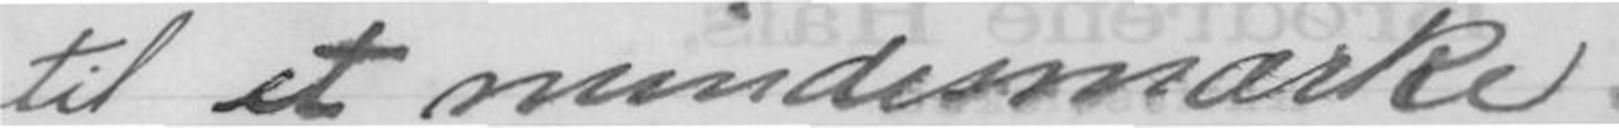til et mindesmærke
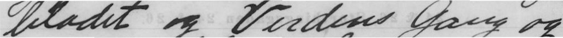bladet og Verdens Gang og
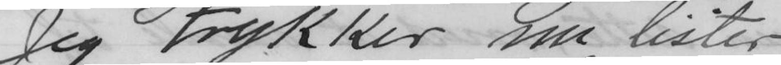Jeg trykker nu lister
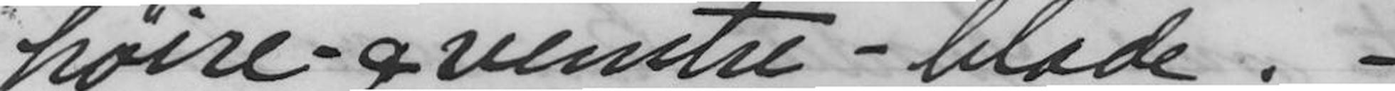høire- & venstre-blade. -
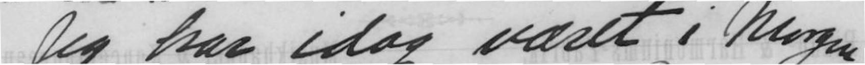Jeg har idag været i Morgen
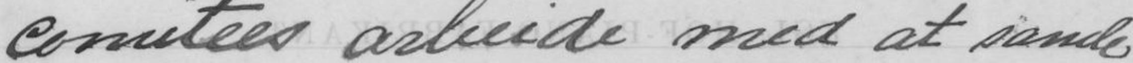comites arbeide med at samle
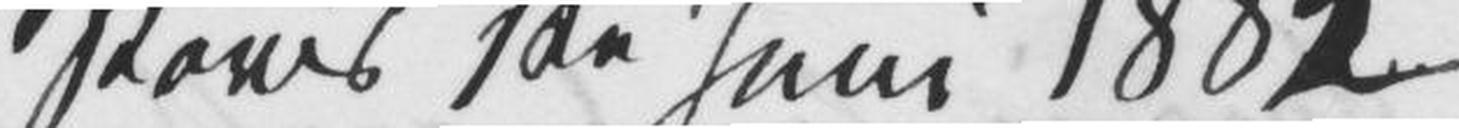Paris 1ste Juni 1882
